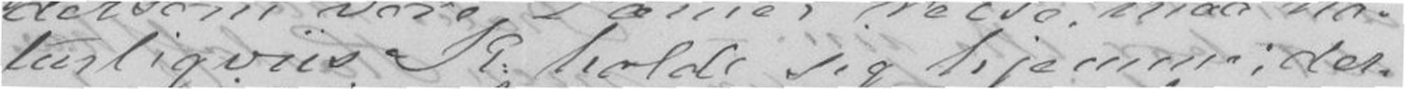turligviis K. holde sig hjemme; der-
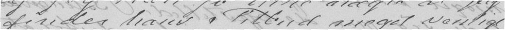findes hans Tilbud meget venligt
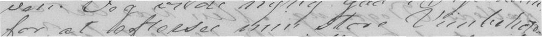for at æfterses min store Viinbehold-
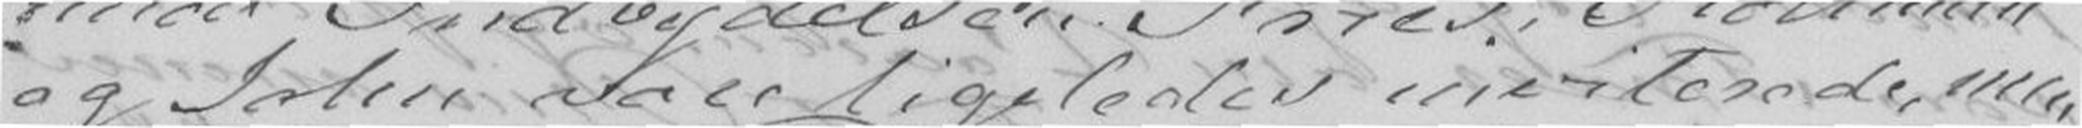og John vare ligeledes inviterede, men
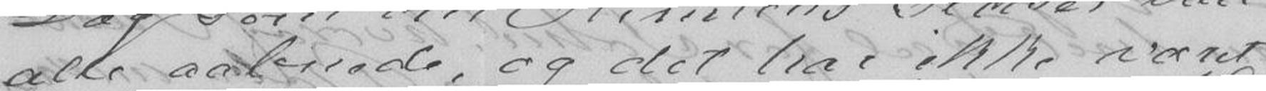alle aabnede, og det har ikke været,
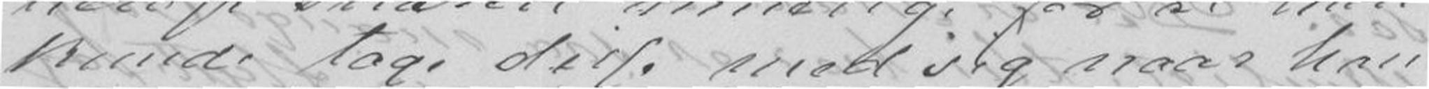kunde tage disse med sig naar han
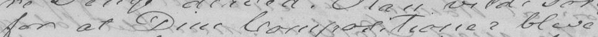for at Dine Compositioner blive
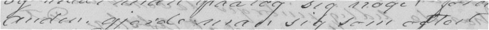Anden gjorde man sig som oftest
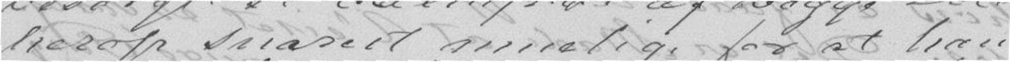herop snarest mulig, for at han
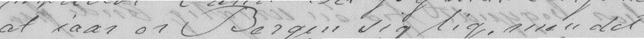at iaar er Bergen sig lig, men det
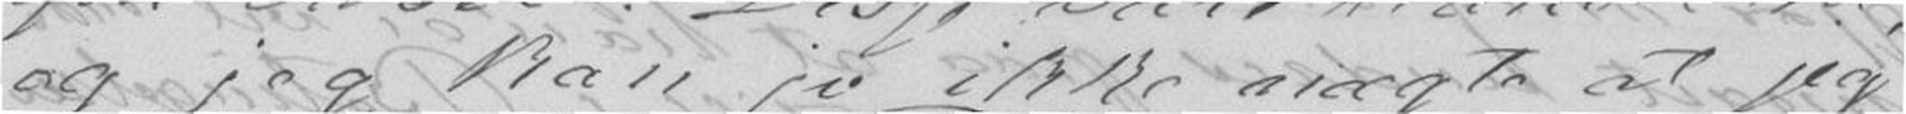og jeg kan jo ikke nægte at jeg
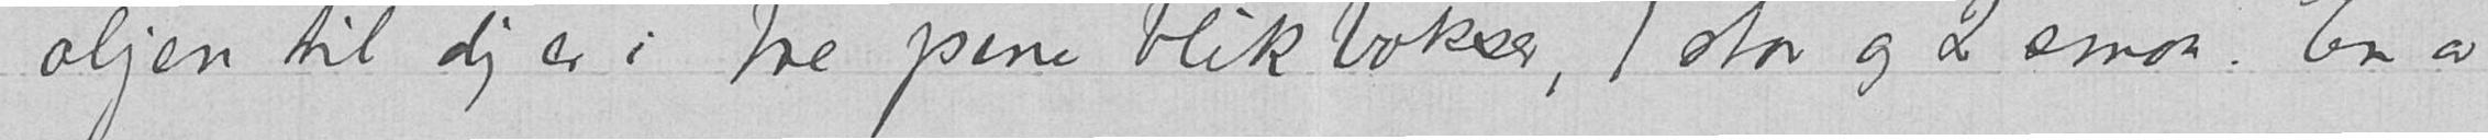oljen til dig er i tre pene blikbokser, 1 stor og 2 smaa. En av
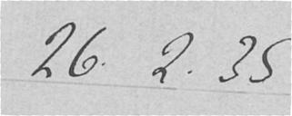26.2.35
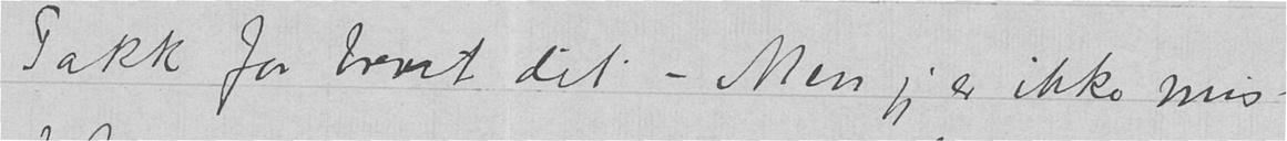Takk for brevet dit – Men jeg er ikke mis-
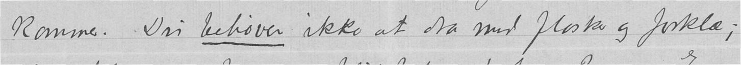kommer. Du behøver ikke at dra med flasker og forklæ;
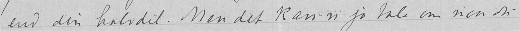end din halvdel. Men det kan vi jo tale om naar du
